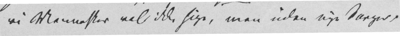vi Mennesker vel ikke sige, men uden nye Sorger.
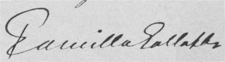Camilla Collett.
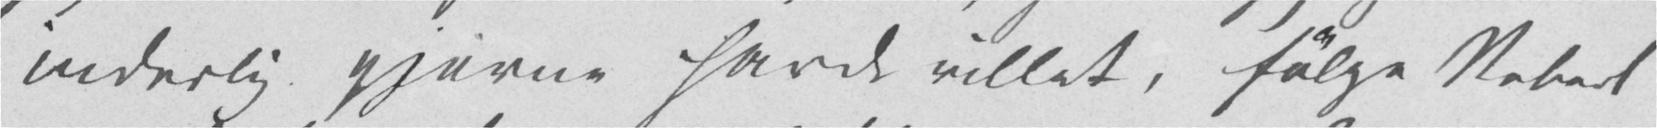inderlig gjerne havde villet, følge Robert
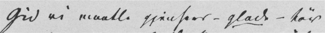Gid vi maatte gjensees – glade – tør
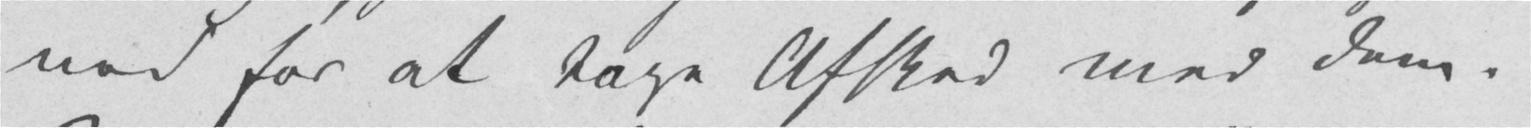ned for at tage Afsked med Dem.
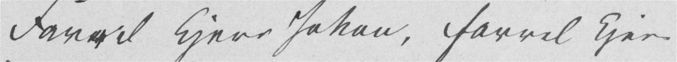Farvel kjere Johan, farvel kjere
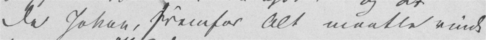De Johan, fremfor Alt maatte vinde
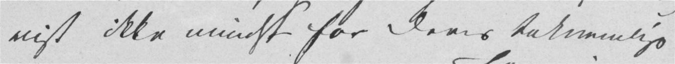vist ikke mindst for Deres taknemlige
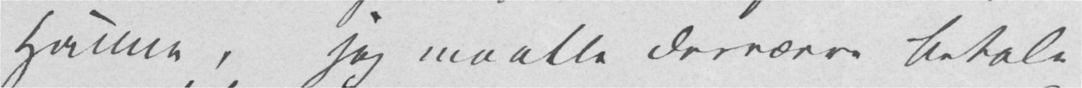Hanne, jeg maatte desværre betale
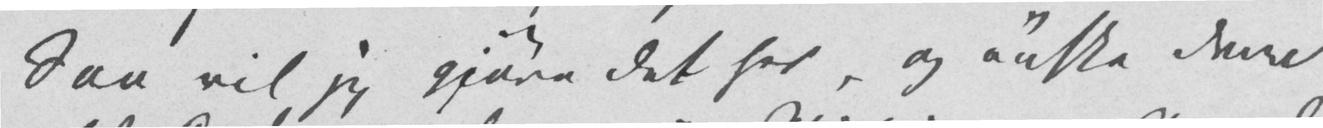Saa vil jeg gjøre det her, og ønske Dere
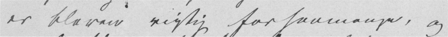er bleven vigtig for saamange, og
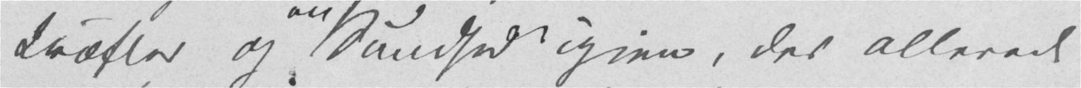Kræfter og Sundhed igjen, der allerede
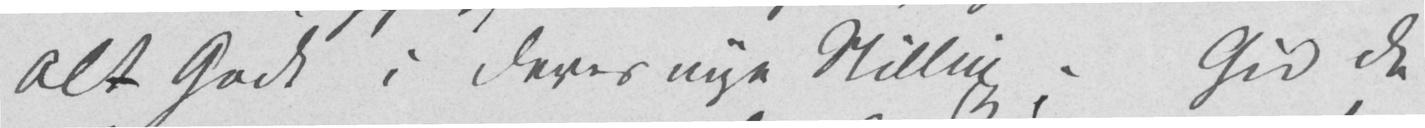Alt Godt i Deres nye Stilling. Gid De
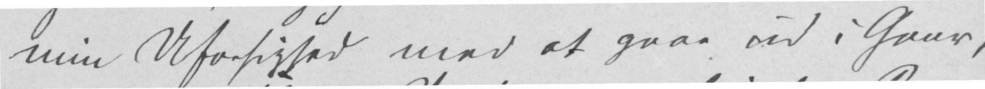min Uforsighed med at gaae ud i Gaar,
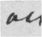en
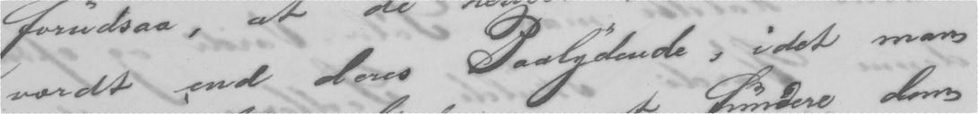værdt end dens Paalydende, idet man
